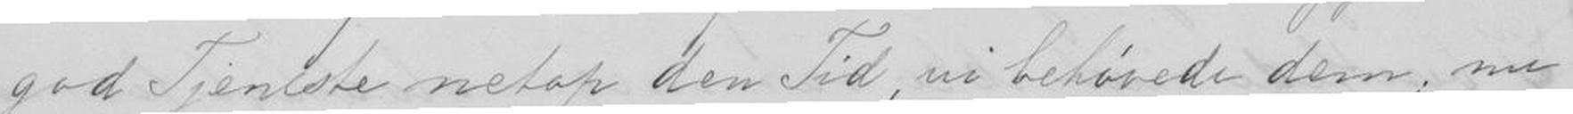god Tjeneste netop den Tid, vi behøvede dem; nu
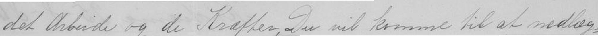det arbeide og de Kræfter, Du vil komme til at medlæg-
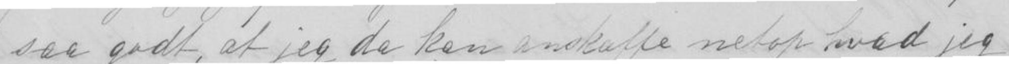saa godt, at jeg de kan anskaffe netop hvad jeg
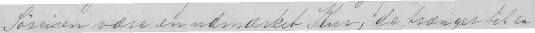Søreisen vore en udmærket kur, de trænges til en
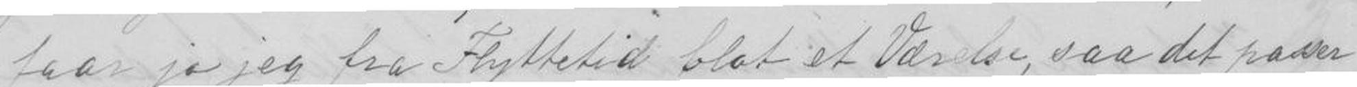faar jeg jo fra Flyttetid blot et værelse, saa det passer
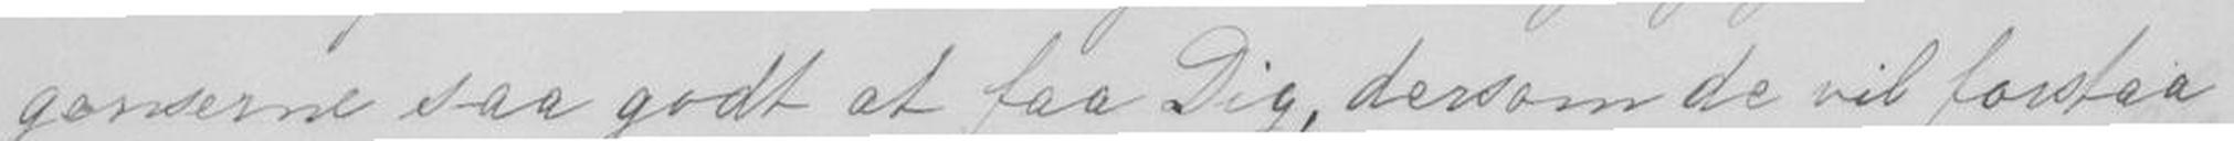gænserne saa godt at faa Dig, dersom de vil forstaa
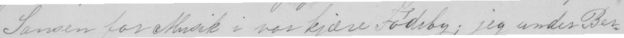Sansen for Musik i vor Kjære Fødeby; jeg under Ber-
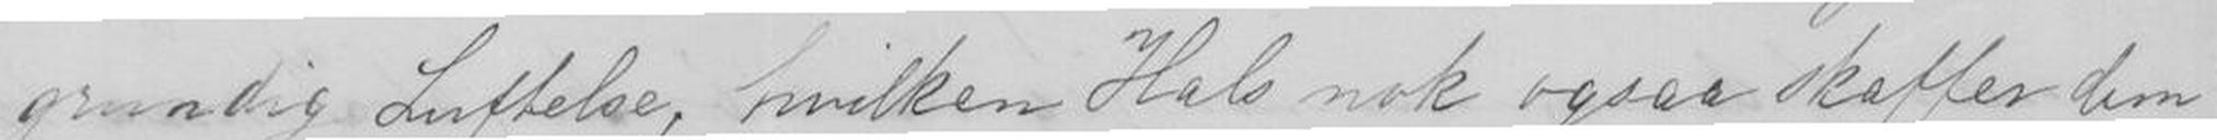grundig Luftelse, hvilken Hals nok ogsaa skaffer dem
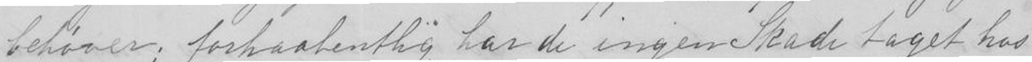behøver; forhaabentlig har de ingen skade taget hos
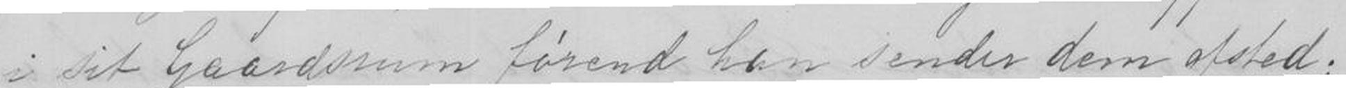i Gaardsrum førend han sender dem afsted;
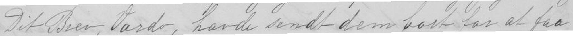Dit Brev, Vardo, havde sendt dem bort for at faa
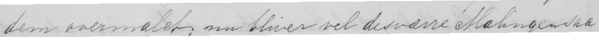dem overmalet; nu bliver vel desværre Malingen saa
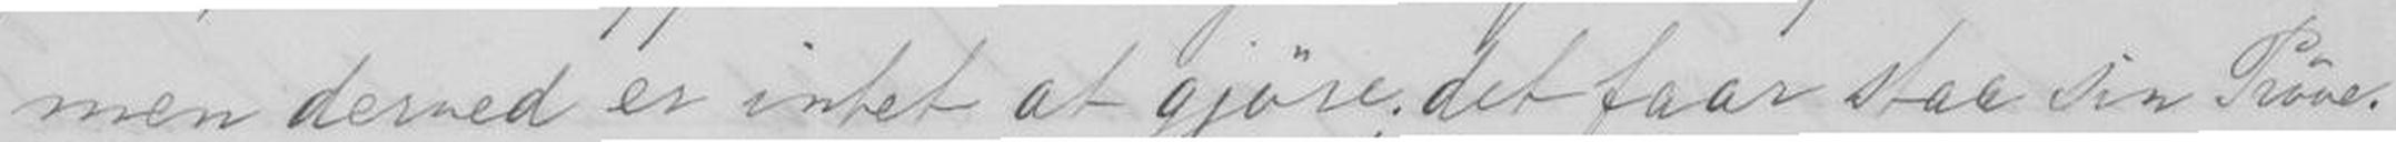med dermed er intet at gjøre; det faar staa Din Prøve.
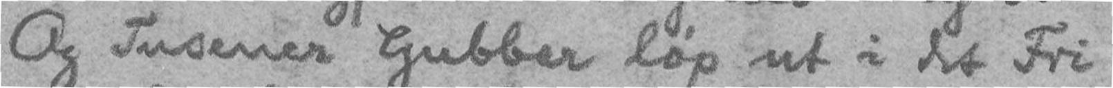Og Tusener Gubber løp ut i det Fri
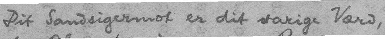Dit Sandsigermot er dit varige Værd,
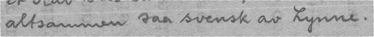altsammen saa svensk av Lynne.
